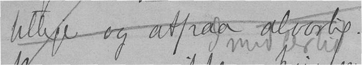billige og atpaa alvorlig.
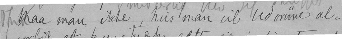ThnnMaa man ikke, hvis man vil bedømme al-
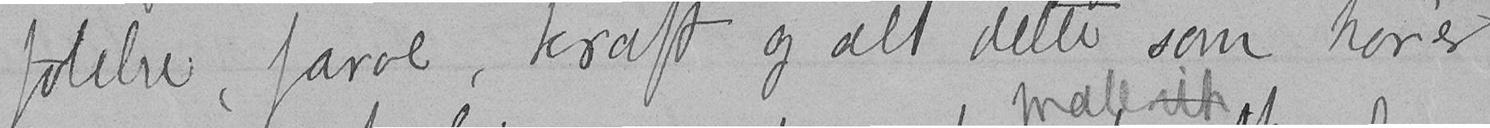følelse, farve, kraft og alt dette som hører
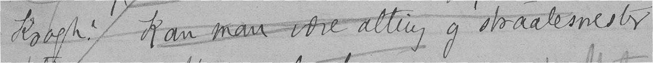Krogh. Kan man være alting og straalesnestig
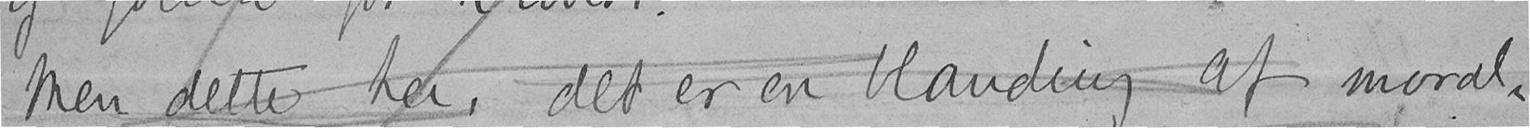Men dette her, det er en blanding af moral-
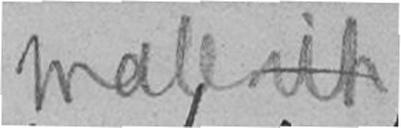malerisk
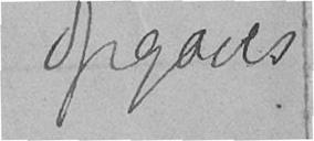opgaves
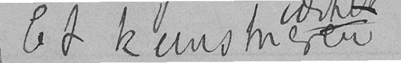Et kunstneren
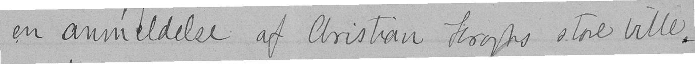en anmeldelse af Christian Kroghs store bille-
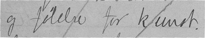og følelse for kunst.
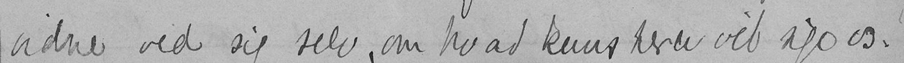vidne ved sig selv, om hvad kunsteren vil sige os.
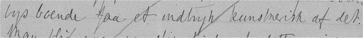bysboende faa et indtryk kunstnerisk af det.
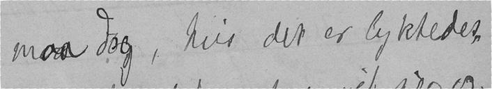maa dog, hvis det er lykkedes
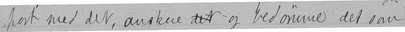port med det, anskue det og bedømme det som
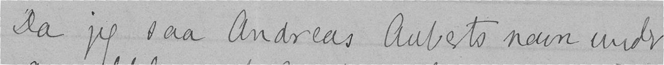Da jeg saa Andreas Auberts navn under
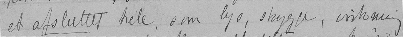et afsluttet hele, som lys, skygge, virkning
